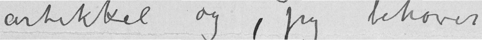artikkel og, jeg behøver
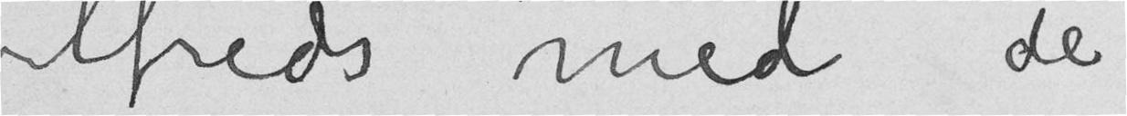tilfreds med de
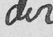der
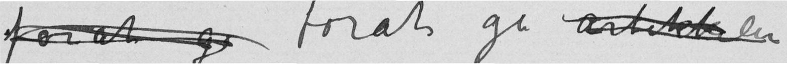forat gi forat gi artikkelen
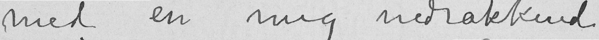med en mig nedrakkende
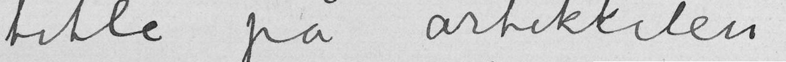title på artikkelen –
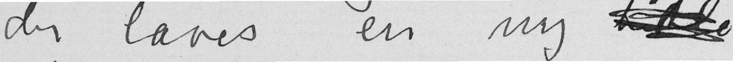der laves en ny title
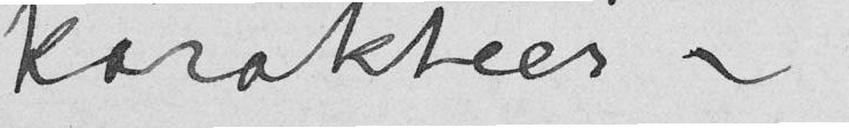karakteer –
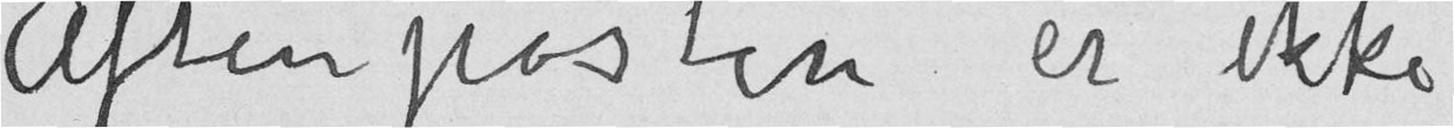Aftenposten er ikke
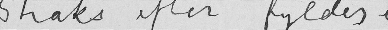Straks efter fyldes i
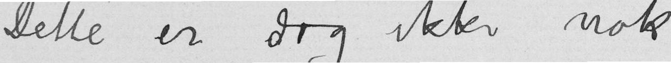Dette er dog ikke nok
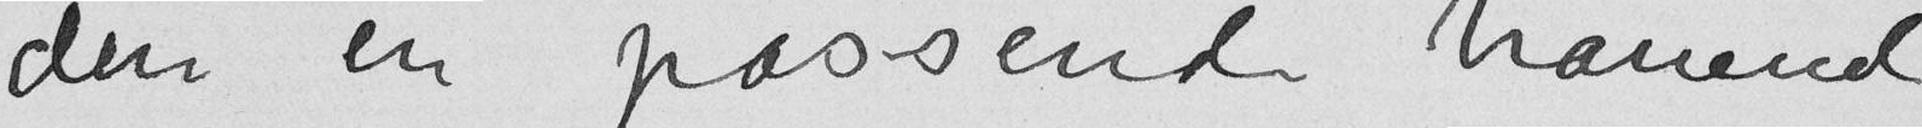den en passende hånende
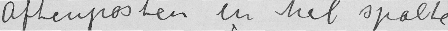Aftenposten en hel spalte
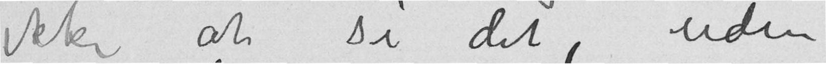ikke at si det, uden
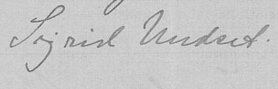Sigrid Undset.
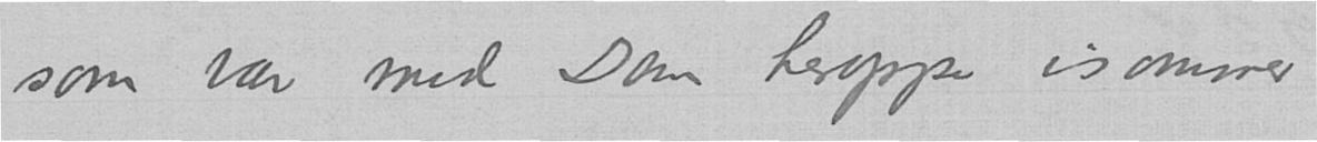som var med Dem heroppe isommer
